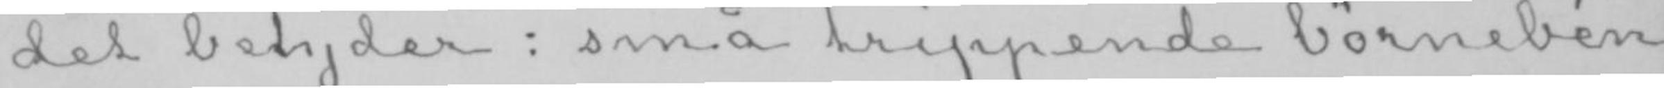det betyder: små trippende børnebén
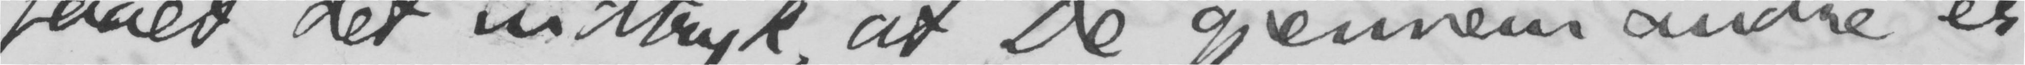faaet det indtryk, at De gjennem andre er
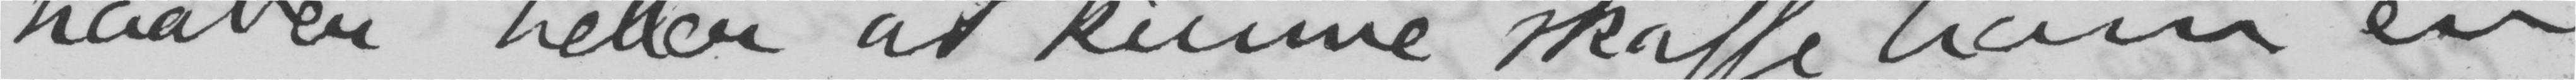haaber heller at kunne skaffe ham en
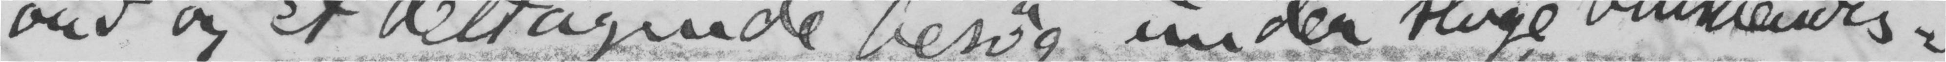ord og et deltagende besøg under slige omstændig-
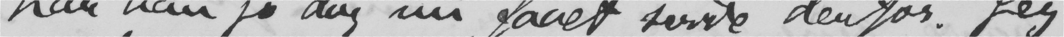har han jo dog nu faaet svide derfor. Jeg
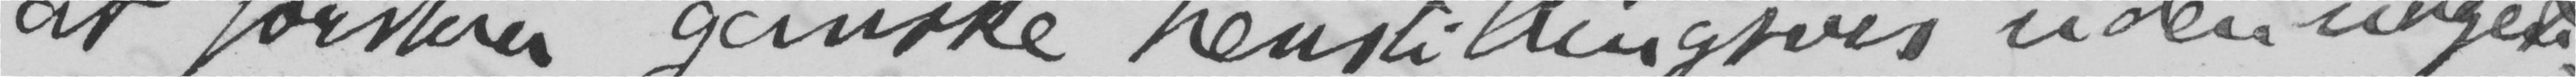at forstaa ganske henstillingsvis uden noget-
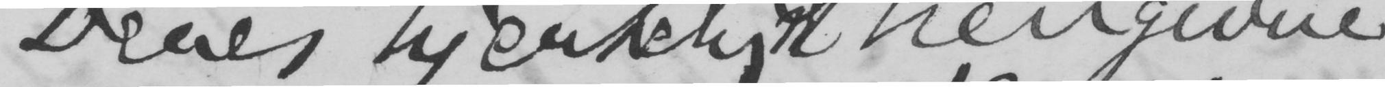Deres hjerteligst hengivne
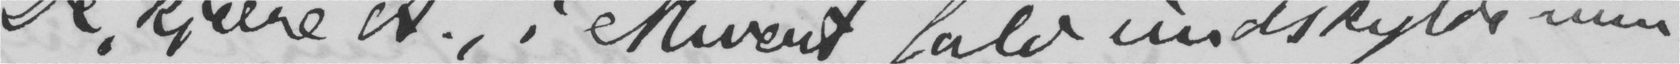De, kjære A., i ethvert fald undskylde min
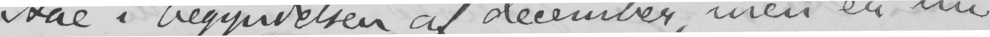Aae i begyndelsen af december, men er nu
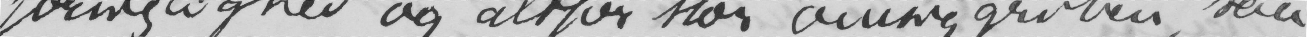forsigtighed og altfor stor omsiggriben, saa
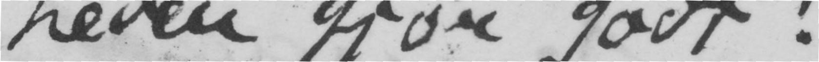heder gjør godt!
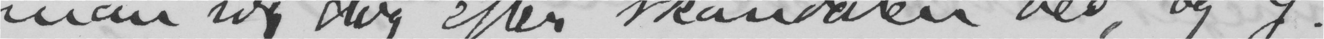man sig dog efter skandalen ved, og G.
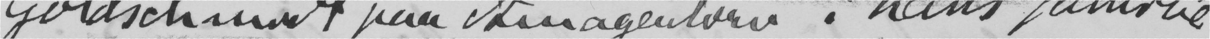Goldschmidt paa Amagertorv, i hans familie
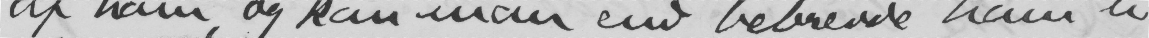af ham, og kan man end bebreide ham u-
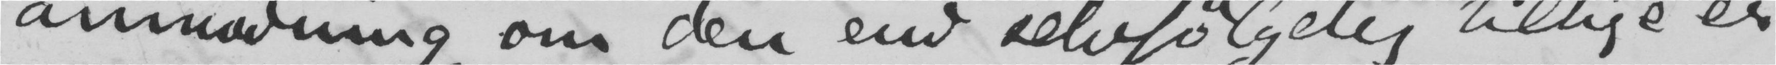anmodning, om den end selvfølgelig tillige er
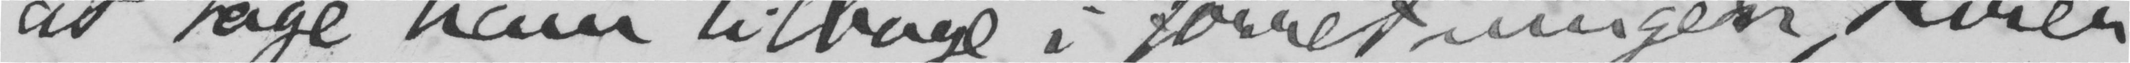at tage ham tilbage i forretningen, kvier
